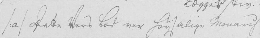/: a / Dette Vers lod vor høysalige Monarch
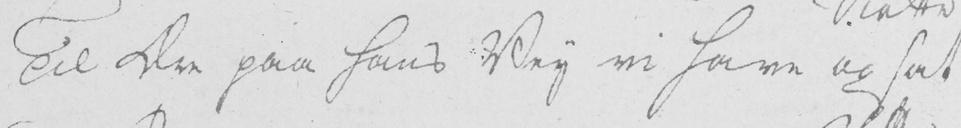Til Ære paa hans Vey vi have opsat
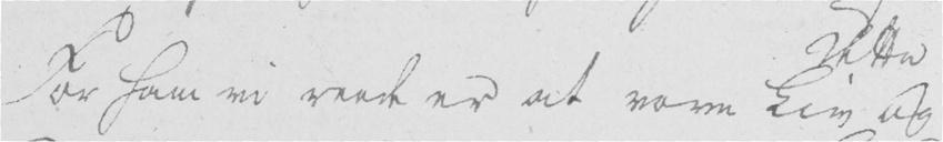For ham vi reede er at vove Liv og
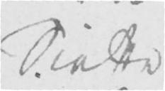Siette
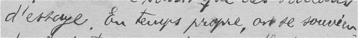d’essage. En temps propre, on se souvien-
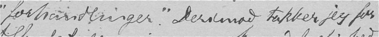"forhandlinger". Derimod takker jeg for
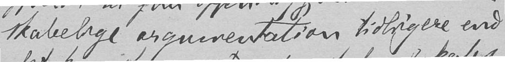skabelige argumentation tidligere end
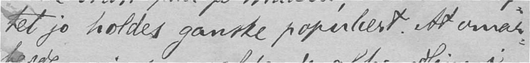tet jo holdes ganske populært. At omar-
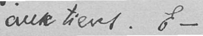aux tiens. E - .
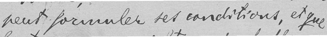pent formuler ses conditions, et que
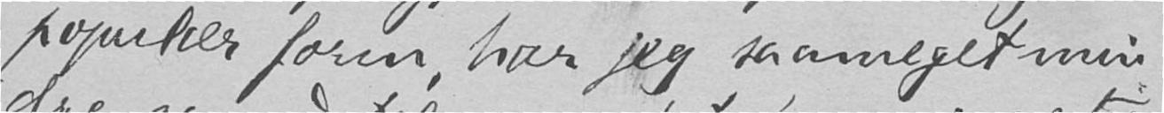populær form har jeg saameget min-
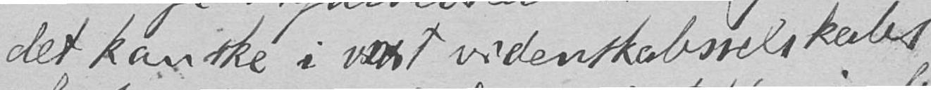det kan ske i vort videnskabsselskabs
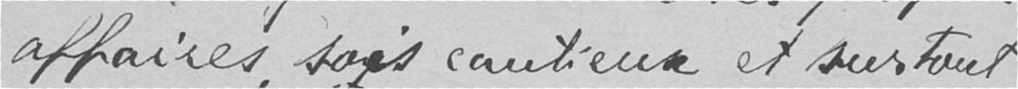affaires, sois cautieus et surtout
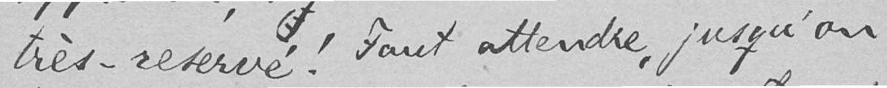très-reservé! Tant attendre, jusqu'on
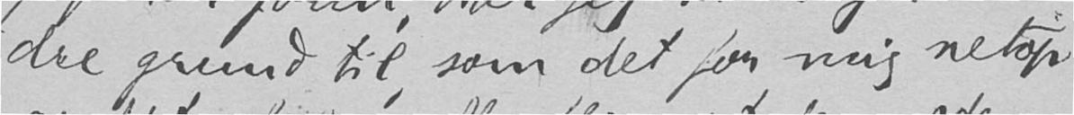dre grund til, som det for mig netop
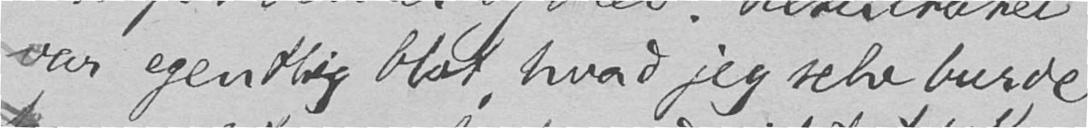var egentlig blot, hvad jeg selv burde
